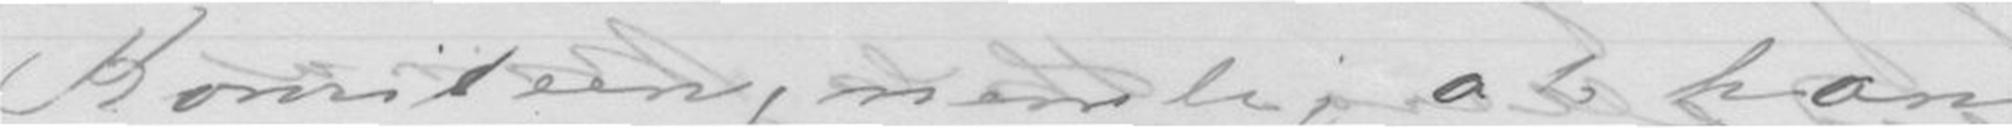Komiteen, nemlig at han
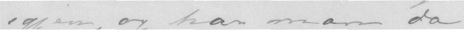igjen, og har man da
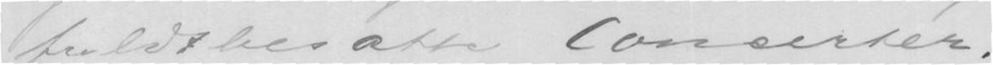fuldt besatte Concerter.
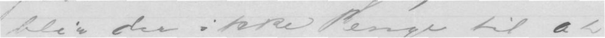blir der ikke Penge til at
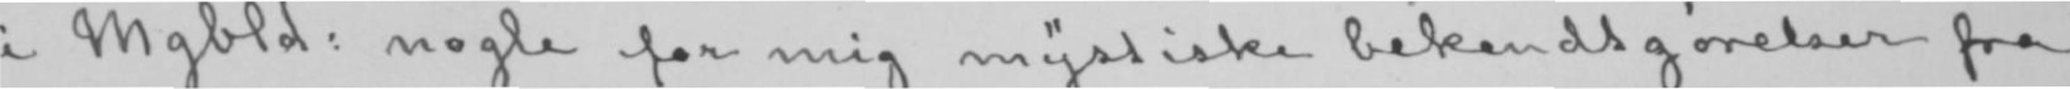i Mgbld: nogle for mig mystiske bekendtgørelser fra
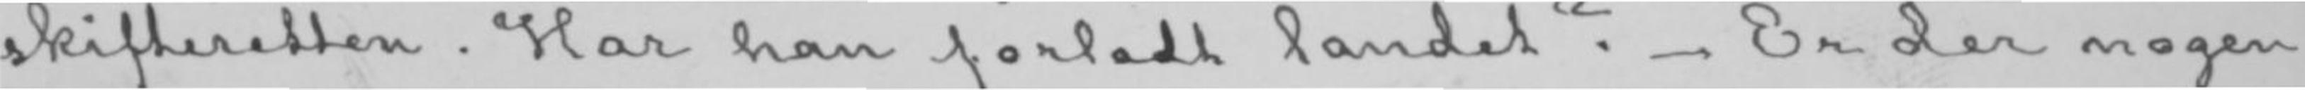skifteretten. Har han forladt landet?- Er der nogen
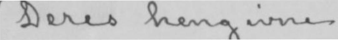Deres hengivne
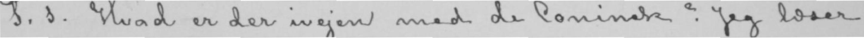P. s. Hvad er der ivejen med de Coninck? Jeg læser
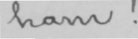ham!
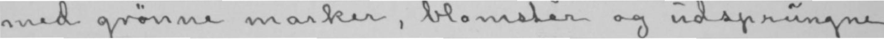med grønne marker, blomster og udsprungne
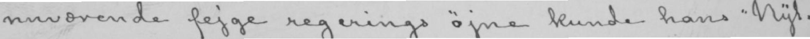nuværende fejge regerings øjne kunde hans «Nyt-
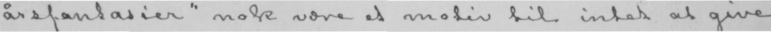årsfantasier» nok være et motiv til intet at give
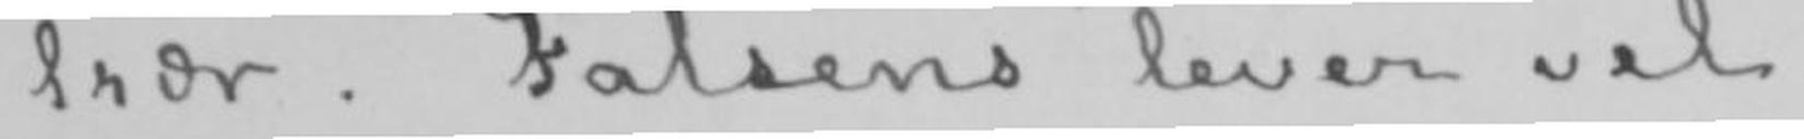trær. Falsens lever vel.
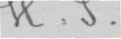H. I.
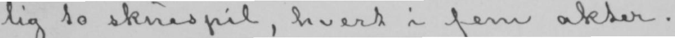lig to skuespil, hvert i fem akter.-
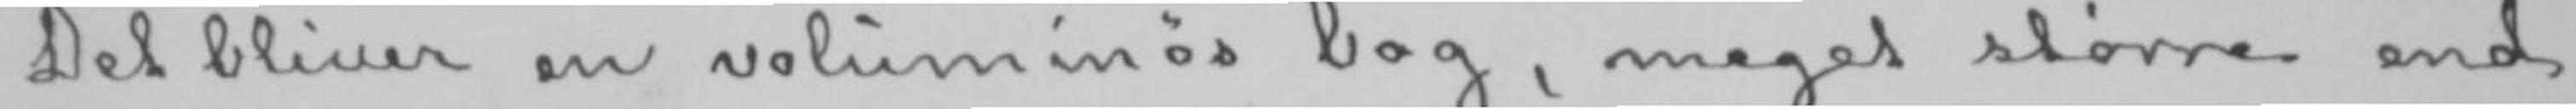Det bliver en voluminøs bog, meget større end
